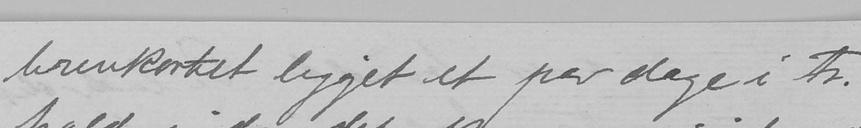brevkortet ligget et par dage i Fr.
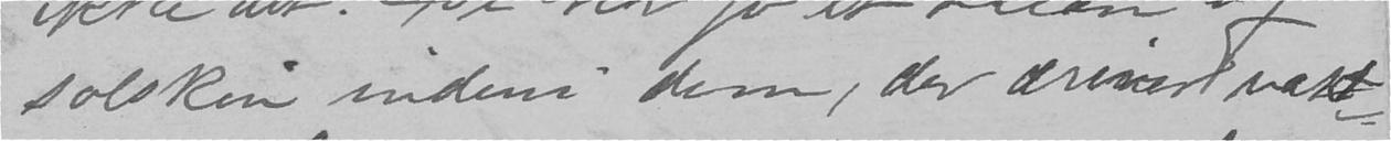solskin indeni dem, der driver væks
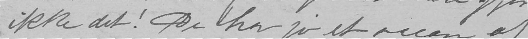ikke det! De har jo et ocean af
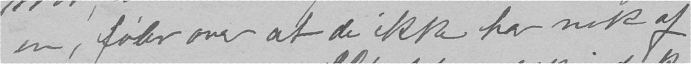en, føler over at de ikke har nok af
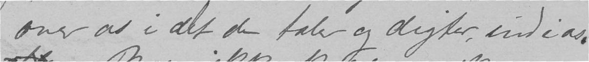over os i det de taler og digter,ind i os.
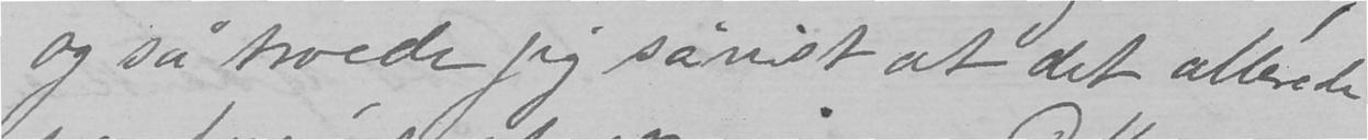og så troede jeg såvidt at det allerede
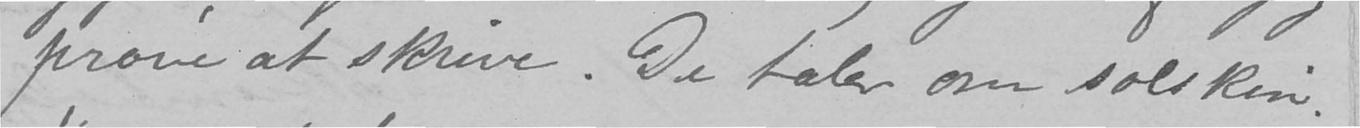prøve at skrive. De taler om solskin:
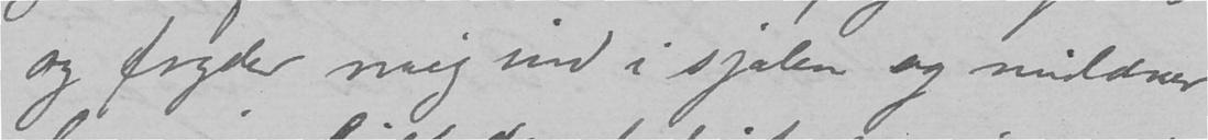og fryder mig ind i sjelen og mildner
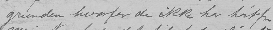grunden hvorfor de ikke har hørt fra
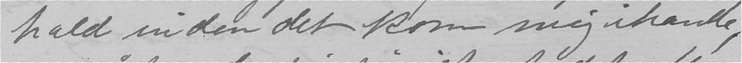hald inden det kom mig ihænde,
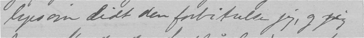ligesom lidt den forbitrelse jeg, og jeg
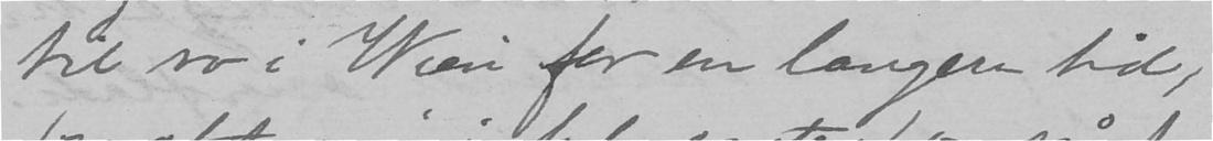til ro i Wien for en længere tid,
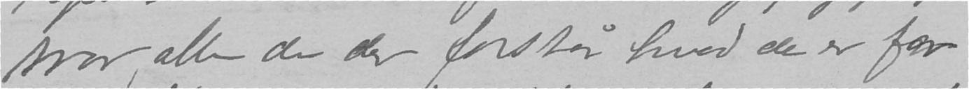tror alle de der forstår hvad de er for
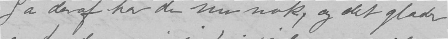Ja deraf har de nu nok, og det glæder
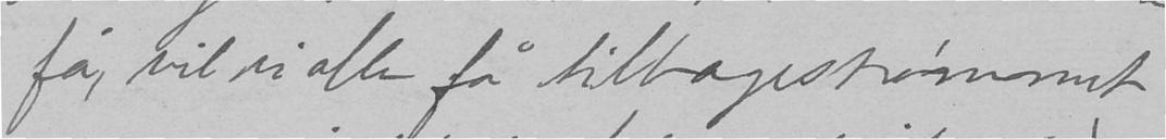få, vil vi alle få tilbagestrømmet
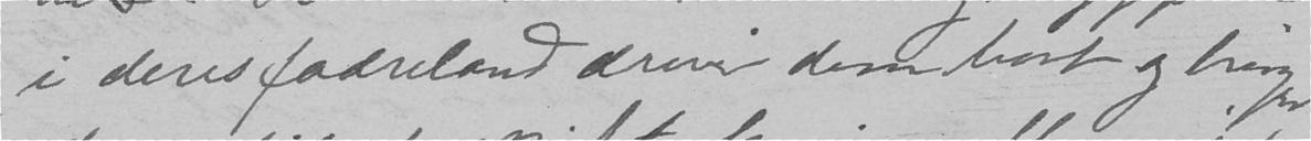i deres fædreland driver dem bort og bringer
